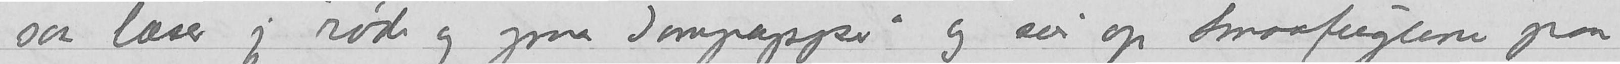saa læser jeg «røde og graa dompapper» og saa sier op Smaafuglene paa
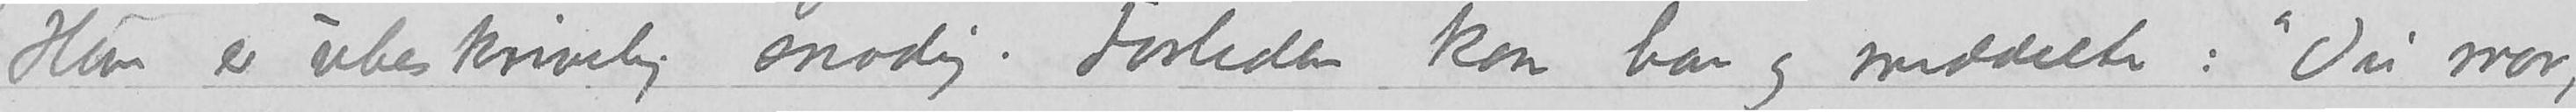Han er ubeskrivelig snodig. Forleden kom han og meddelte: «Du mor,
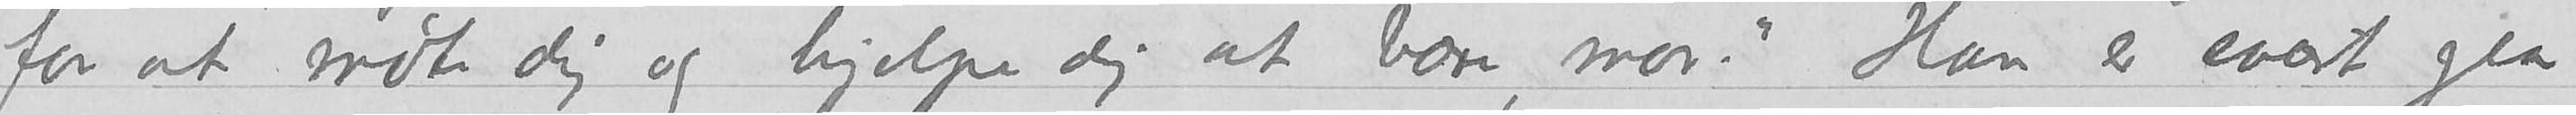for at møte dig og hjelpe dig at bære, mor.»  Han er svært gla
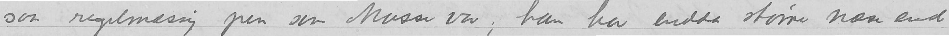saa regelmæssig pen som Mosse var; han har endda større næse end
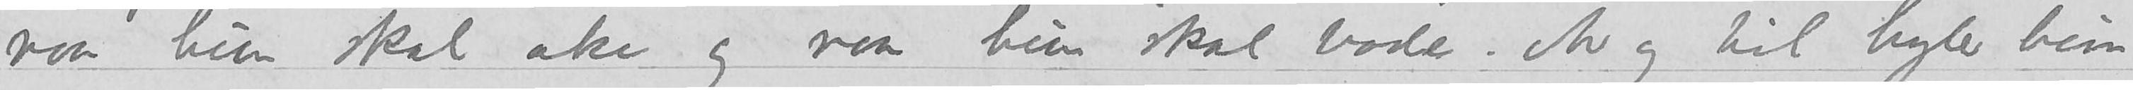naar hun skal ake og naar hun skal bade.  Av og til hyler hun
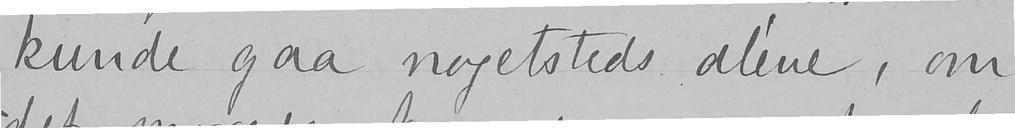kunde gaa nogetsteds alene, om
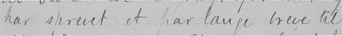har skrevet et par lange breve til
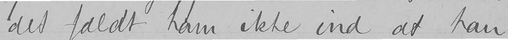det faldt ham ikke ind, at han
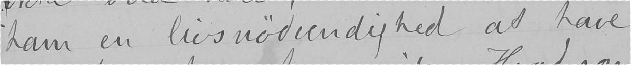ham en livsnødvendighed at have
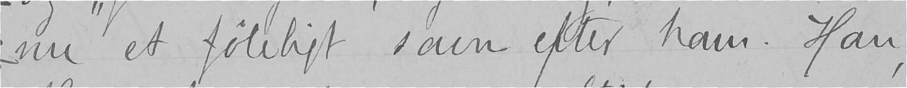nu et føleligt savn efter ham. Han,
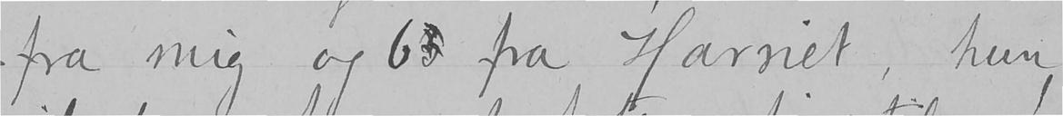fra mig og 65 fra Harriet, hun
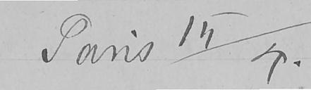Paris 15/4.
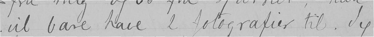vil bare have 2 fotografier til. Jeg
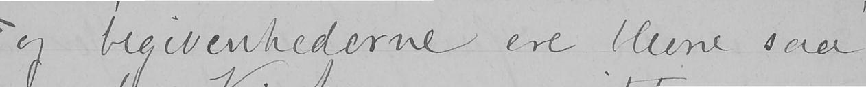og begivenhederne ere blevne saa
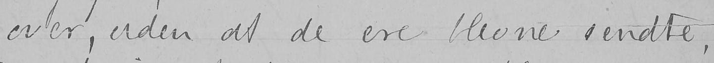over, uden at de ere blevne sendte,
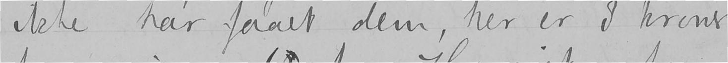ikke har faaet dem, her er 9 kroner
